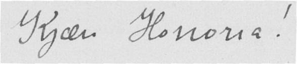Kjære Honoria!
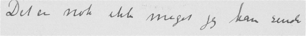Det er nok ikke meget jeg kan sende
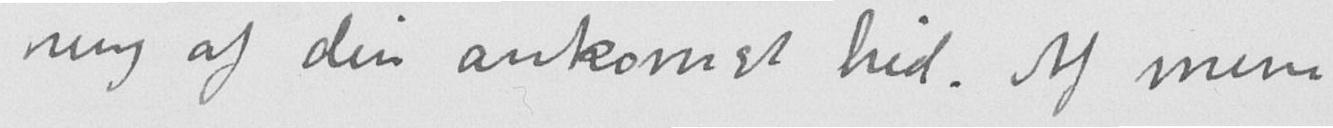ning af din ankomst hid. Af mine
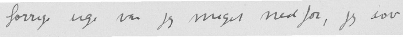forrige uge var jeg meget nedfor, jeg sov
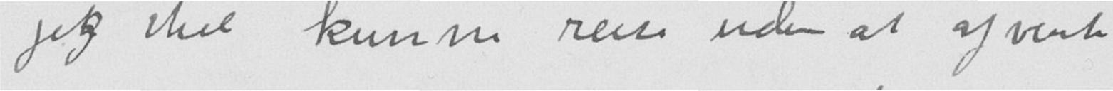jeg skal kunne reise uden at afvente
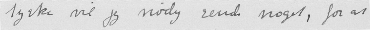tyske vil jeg nødig sende noget, for at
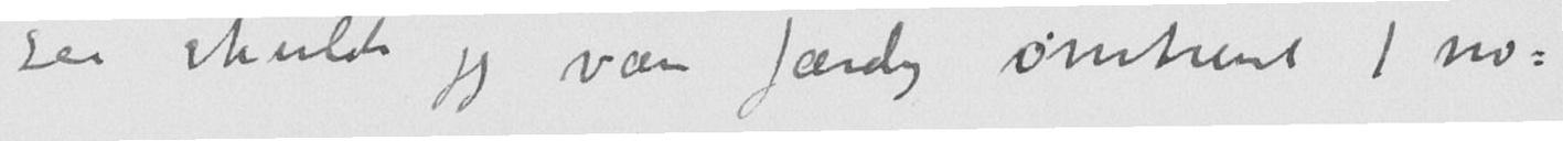saa skulde jeg være færdig omtrent 1 no-
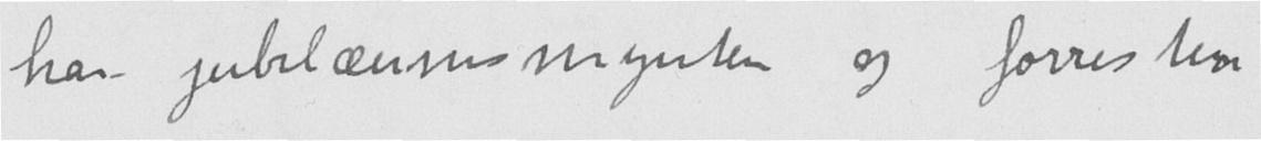har jubilæumsmynter og forresten
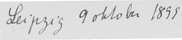Leipzig 9 oktober 1899
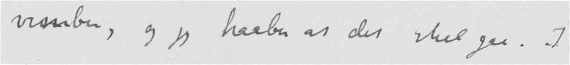vember, og jeg haaber at det skal gaa. I
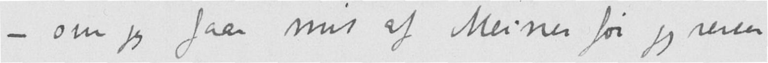- om jeg faar mit af Meiner før jeg reiser
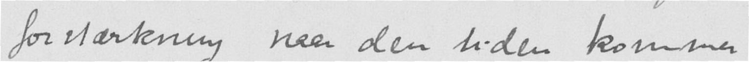forstærkning naar den tiden kommer
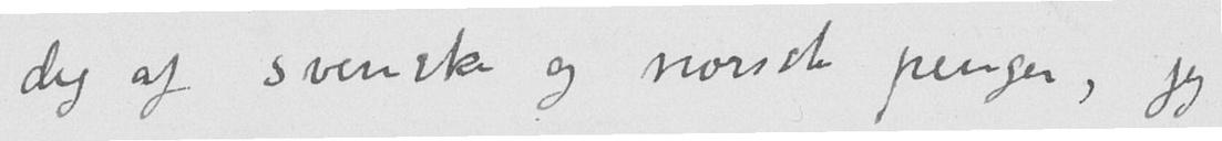dig af svenske og norske penger, jeg
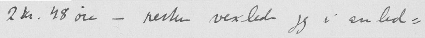2 kr. 48 øre - resten vexlede jeg i anled-
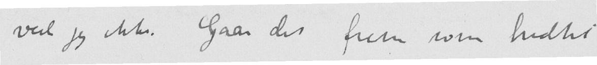ved jeg ikke. Gaar det frem som hidtil
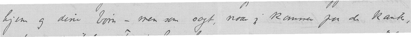hjem og dine børn - men som sagt, naar jeg kommer paa de kanter,
